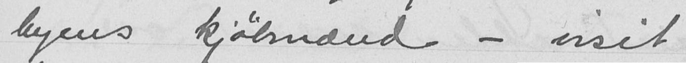byens kjøbmænd - visit
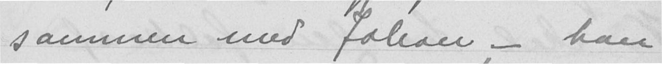sammen med Johan - han
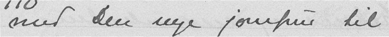med den nye jomfrue til
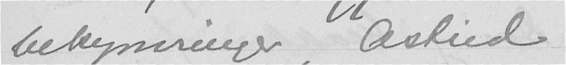bekymringer, Astrid
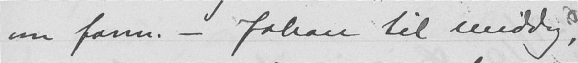om form. - Johan til middag,
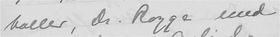boller, Dr. Rogge med
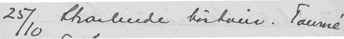25/10 Straalende høstveir. Tourné
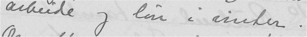arbeide og løn i vinter.
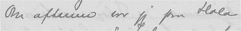Om aftenen var jeg paa Hola
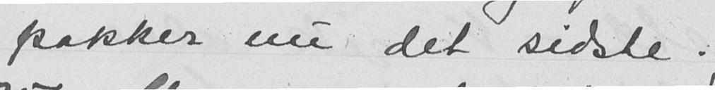pakker nu det sidste.
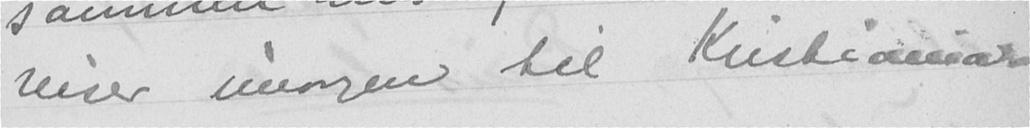reiser imorgen til Kristiania.
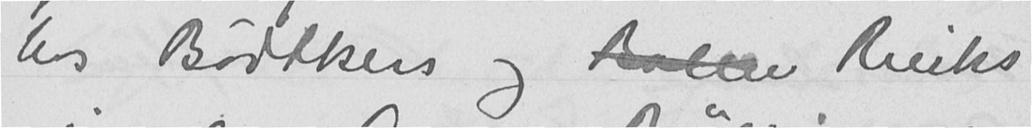hos Bødtkers og Bolme Riecks
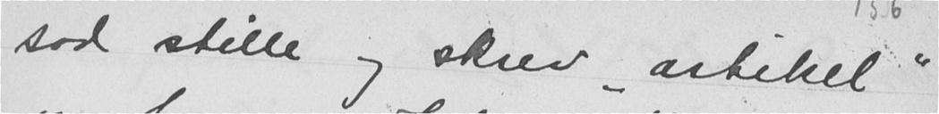sad stille og skrev "artikel"
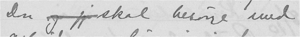Don og jeg skal besørge med
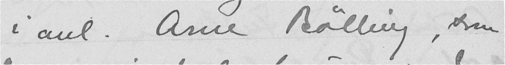i anl. Arne Bølling, som
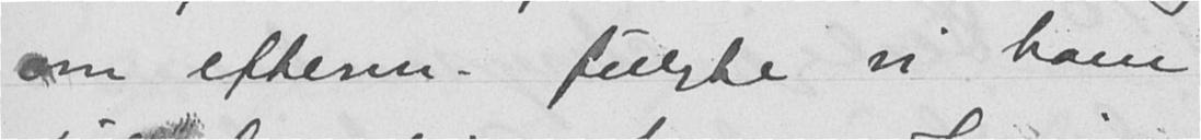om efterm. fulgte vi ham
